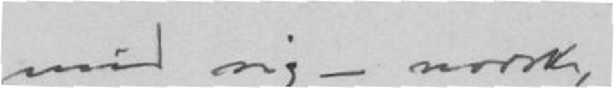med mig - norske,
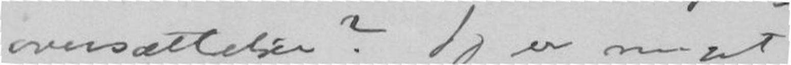oversættelse ? Jeg er nu lit
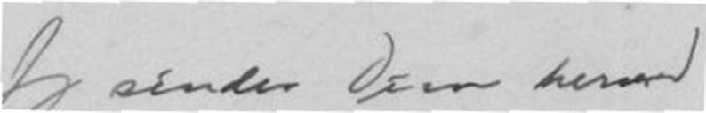Jeg sender Dem hermed
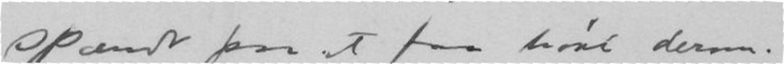spændt paa at faa høre derom.
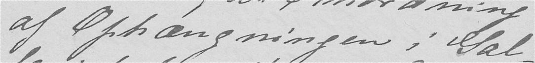af Ophængningen i Gal-
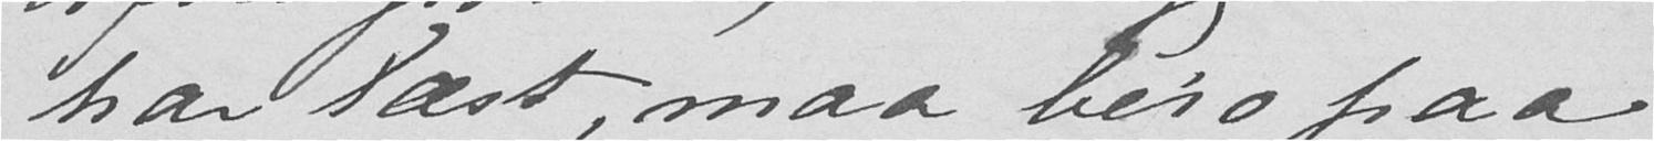har læst, maa bero paa
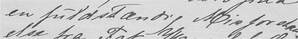en fuldstændig Misforstaa-
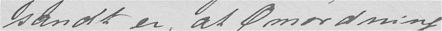sandt er, at Omordning
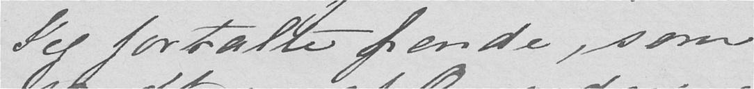Jeg fortalte hende, som
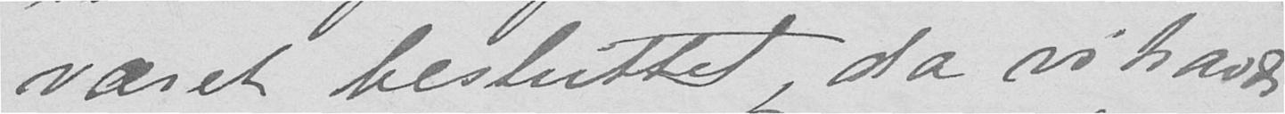været besluttet, da vi havde
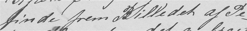finde frem Billedet af Pe-
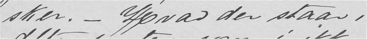sker. - Hvad der staar i
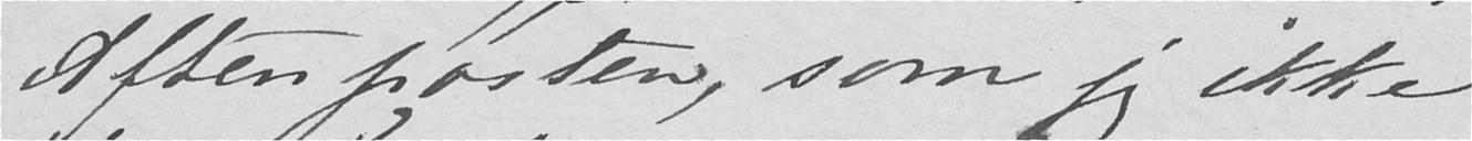Aftenposten, som jeg ikke
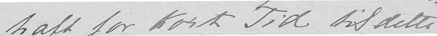haft for kort Tid til dette
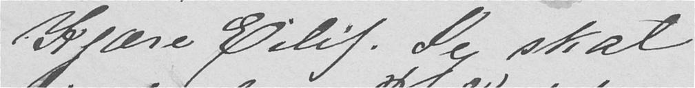Kjære Eilif. Jeg skal
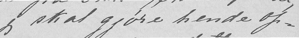og jeg skal gjøre hende op-
